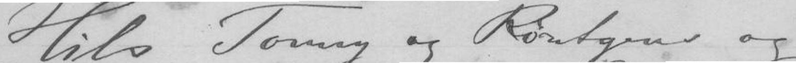Hils Tonny og Röntgens og
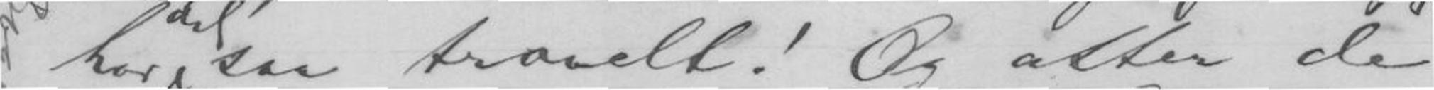har det saa travelt! Og atter de
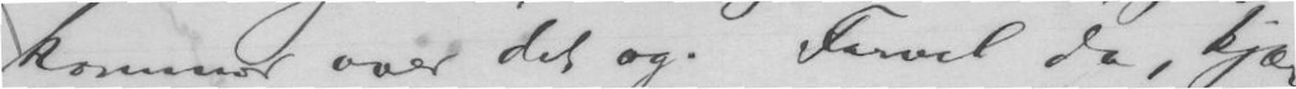kommer over det og. Farvel da, kjære
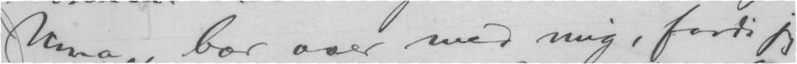Nina, bær over med mig, fordi jeg
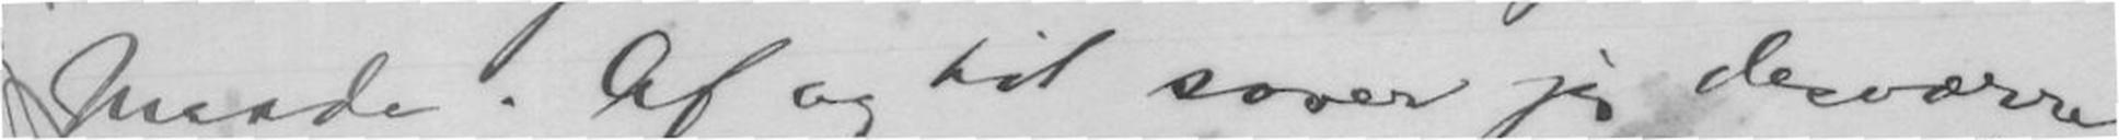Maade. Af og til sover jeg desværre
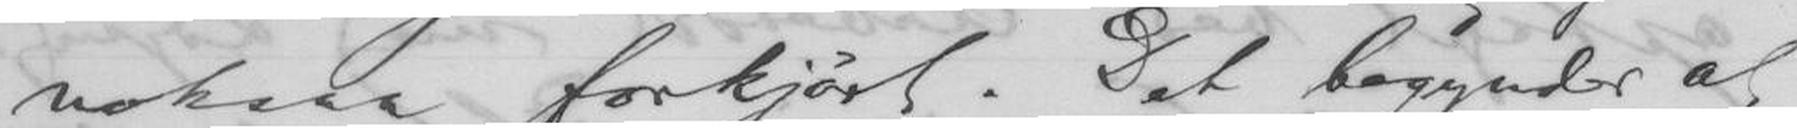noksaa forkjørt. Det begynder at
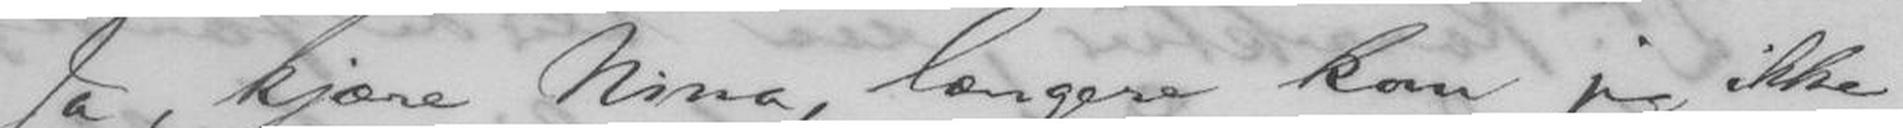Ja, Kjære Nina, længere kom jeg ikke
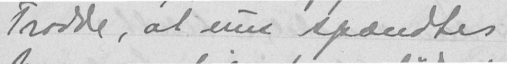Trodde, at nu spændtes
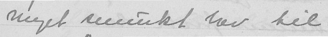meget smukt brev til
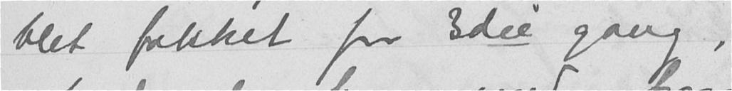blit fakket for 3die gang,
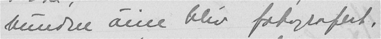bundne øine blev fotografert,
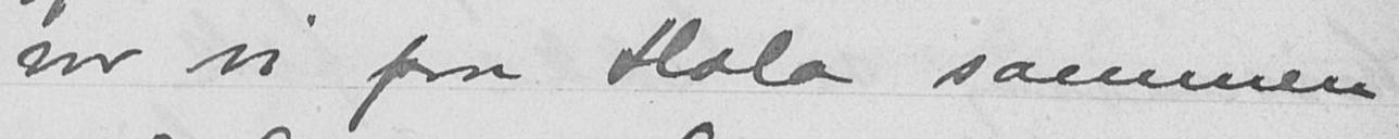var vi paa Hola sammen
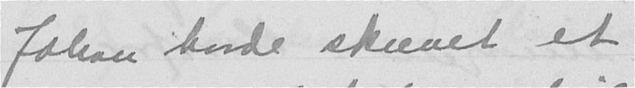Johan havde skrevet et
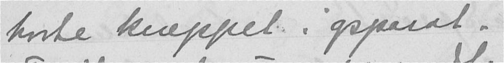hørte kneppet i apparat.
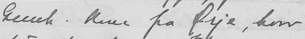Gunh. kom fra Ørje, hvor
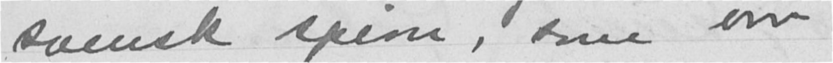svensk spion, som var
